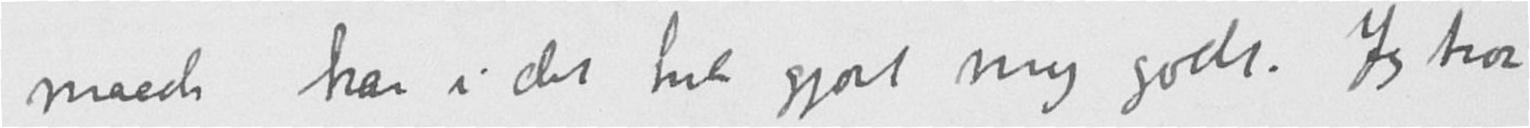maade har i det hele gjort mig godt. Jeg tror
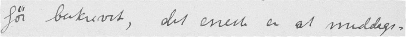før beskrevet, det eneste er at middags-
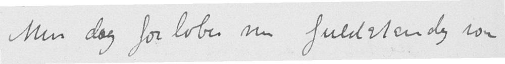Min dag forløber nu fuldstændig som
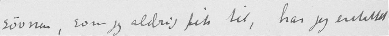søvnen, som jeg aldrig fik til, har jeg erstattet
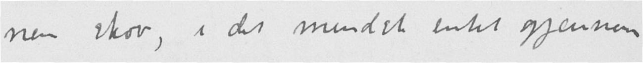nem skov, i det mindste intet gjennem
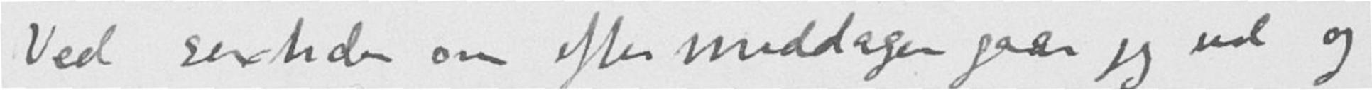Ved sextiden om eftermiddagen gaar jeg ud og
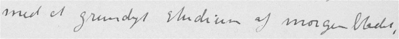med et grundigt studium af morgenbladet,
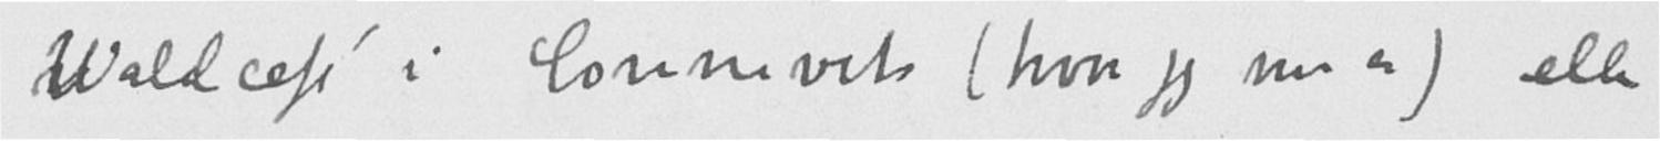Waldcafé i Connevits (hvor jeg nu er) eller
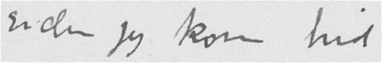siden jeg kom hid
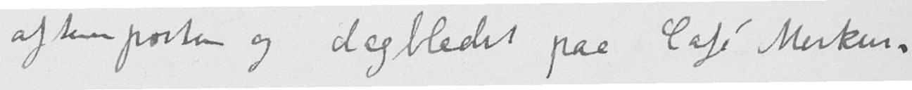aftenposten og dagbladet paa café Merkur.
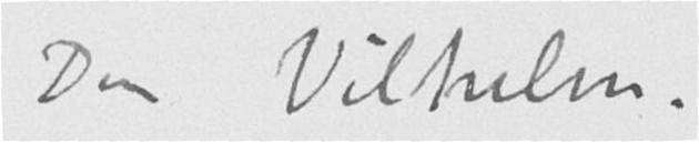Din Vilhelm.
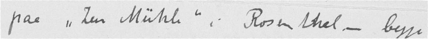paa "Zen Mühle" i Rosenthal - begge
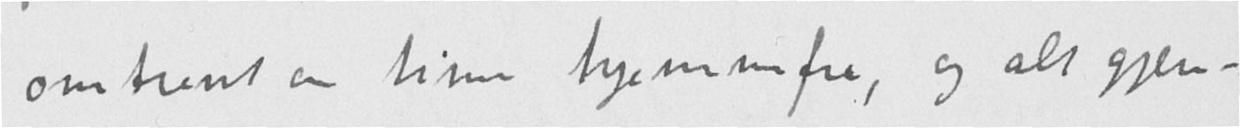omtrent en time hjemmefra, og alt gjen-
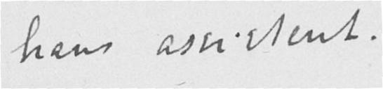hans assistent.
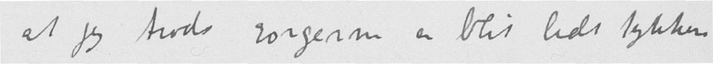at jeg trods sorgerne er blit lidt tykkere
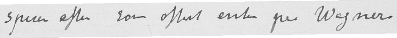spiser aften som oftest enten paa Wagners
